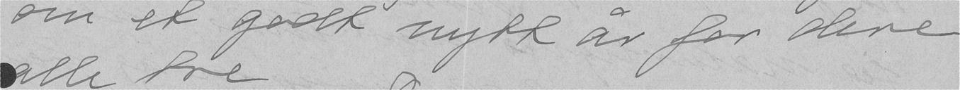om et godt nytt år for dere
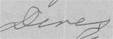Deres
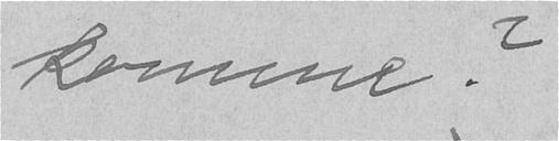komme?
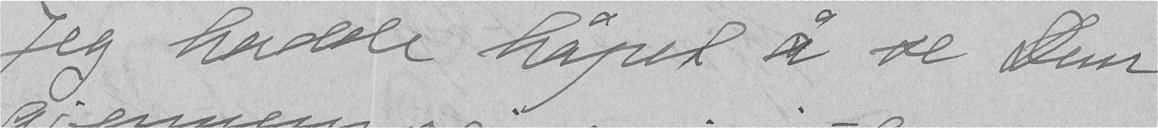Jeg hadde håpet å se Dem
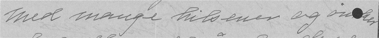Med mange hilsener og ønsker
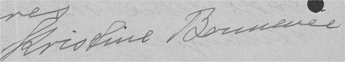Kristine Bonnevie
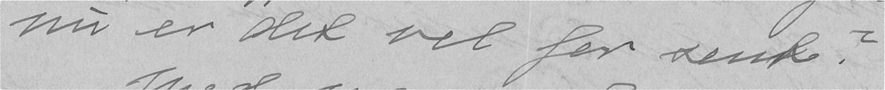nu er det vel for sent?
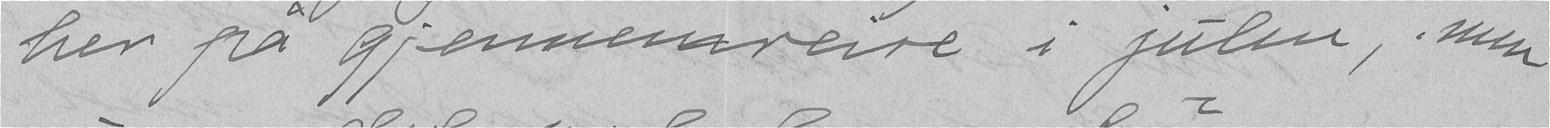her på gjennomreise i julen, men
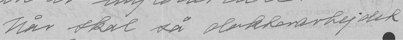Når skal så slakterarbejdet
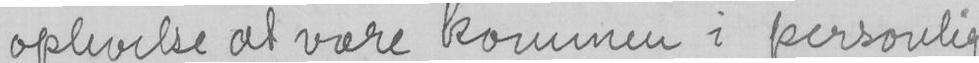oplevelse at være kommen i personlig
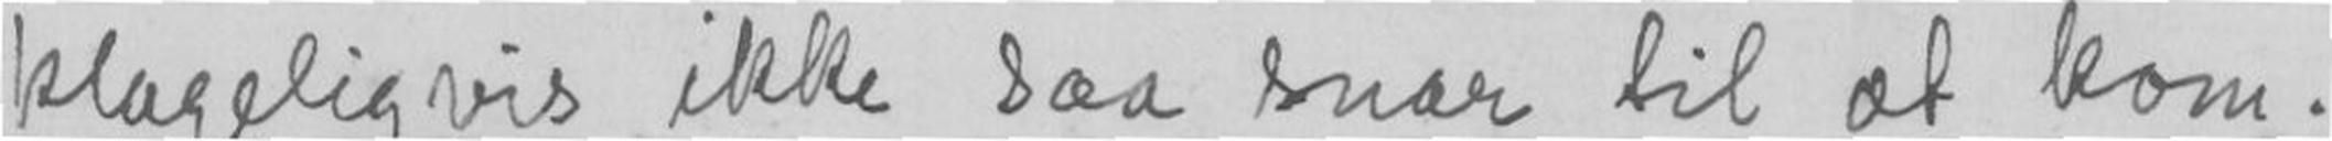klageligvis ikke saa snar til at kom-
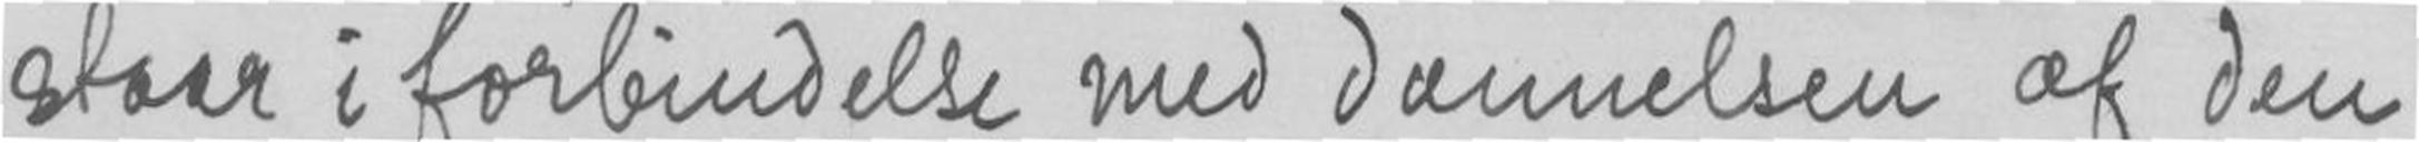staar i forbindelse med dannelsen af den
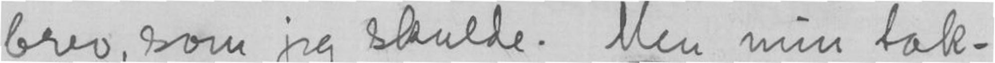brev, som jeg skulde. Men min tak-
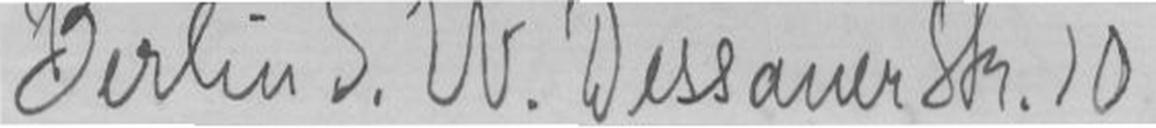Berlin S.W. Dessaner Str. 10
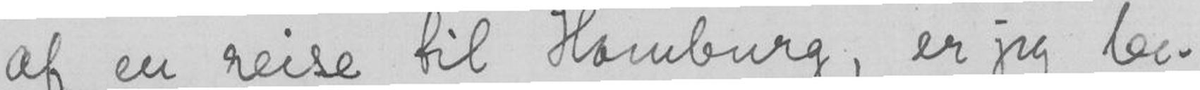af en reise til Hamburg, er jeg be-
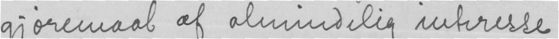gjøremaal af almindelig interesse
